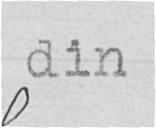din
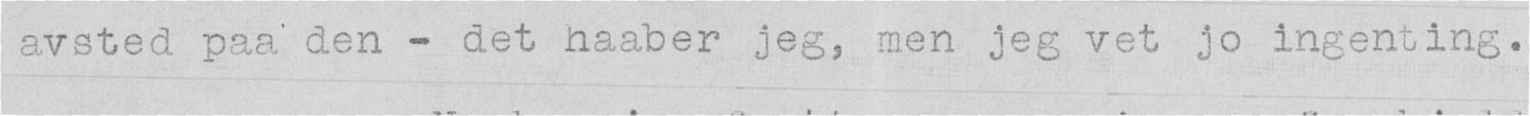avsted paa den - det haaber jeg, men jeg vet jo ingenting.
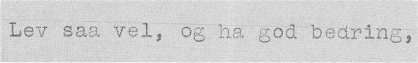Lev saa vel, og ha god bedring,
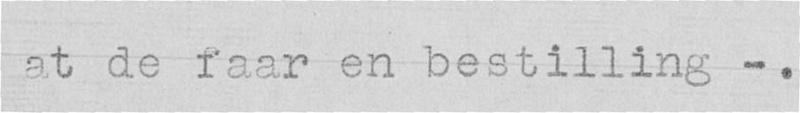at de faar en bestilling -.
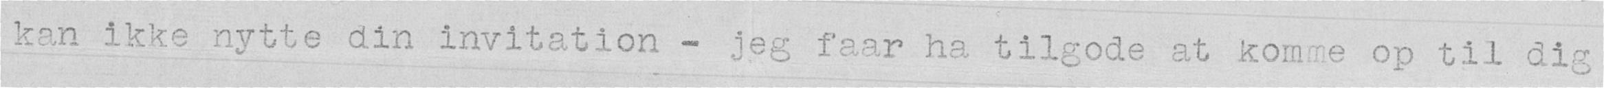kan ikke nytte din invitation - jeg faar ha tilgode at komme op til dig
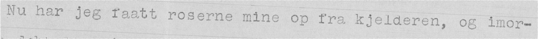Nu har jeg faatt roserne mine op fra kjelderen, og imor-
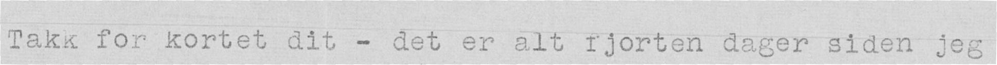Takk for kortet dit - det er alt fjorten dager siden jeg
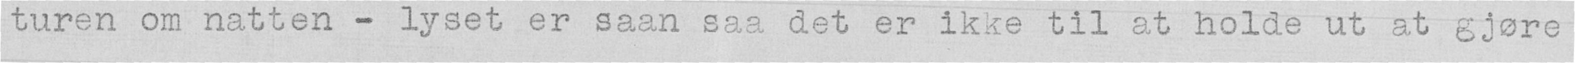turen om natten - lyset er saan saa det er ikke til at holde ut at gjøre
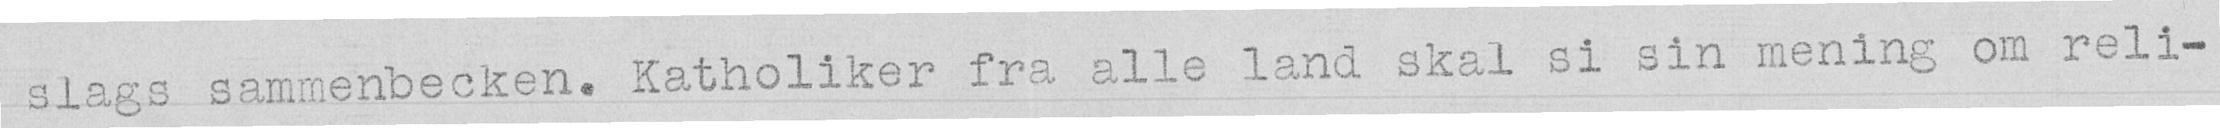slags sammenbecken. Katholiker fra alle land skal si sin mening om reli-
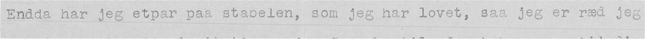Endda har jeg etpar paa stabelen, som jeg har lovet, saa jeg er ræd jeg
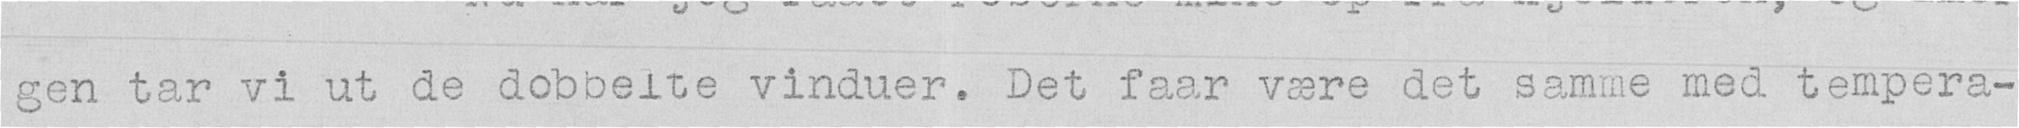gen tar vi ut de dobbelte vinduer. Det faar være det samme med tempera-
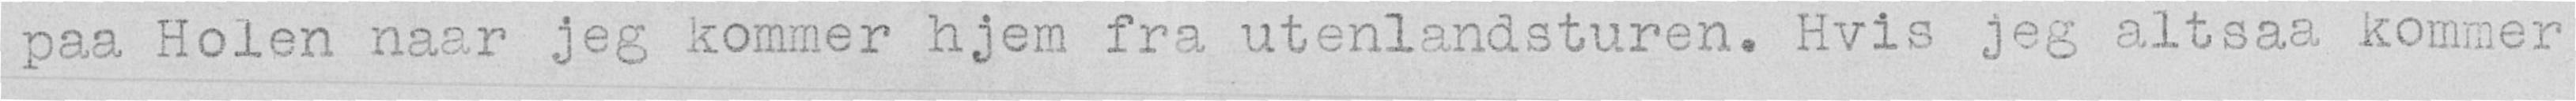paa Holen naar jeg kommer hjem fra utenlandsturen. Hvis jeg altsaa kommer
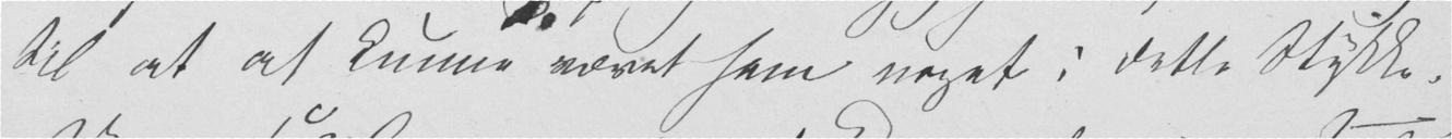til at at kunne været ham noget i dette Stykke.
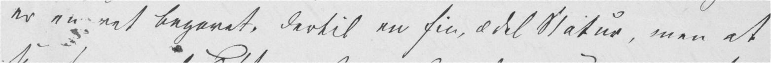er en ret begavet. dertil en fin, ædel Natur, men at
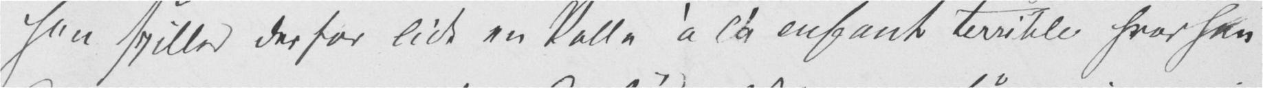han spiller derfor lidt en Rolle á lá enfant terrible hvor han
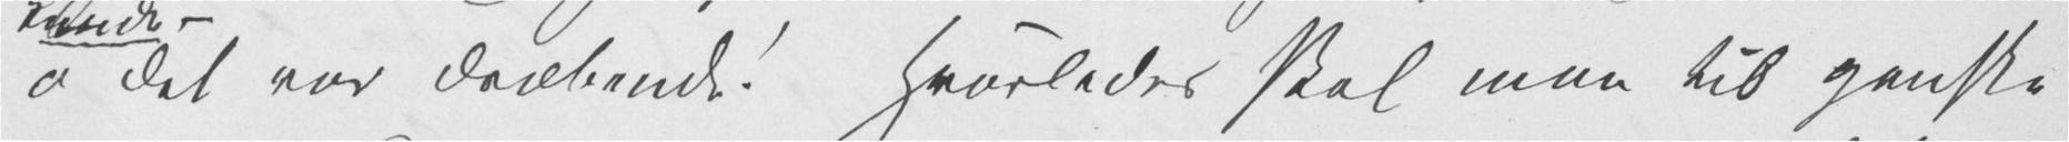o det var dræbende! Hvorledes skal man til ganske
